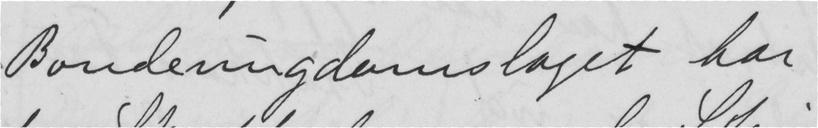Bondeungdomslaget har
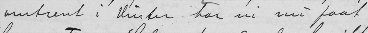omtrent i Vinter har vi nu faat
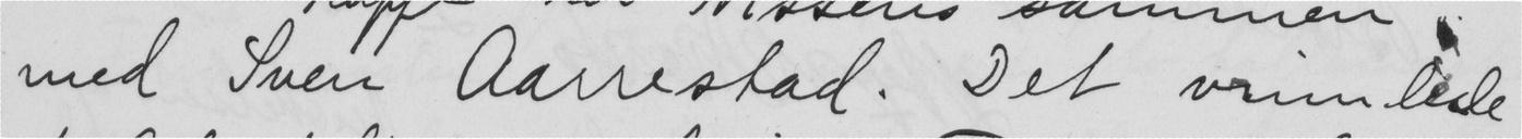med Sven Aanestad. Det vimlede
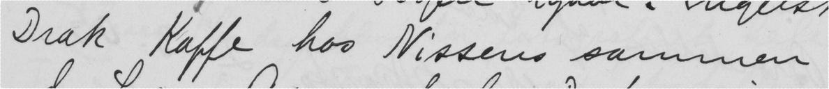Drak Kafle hos Nissens sammen
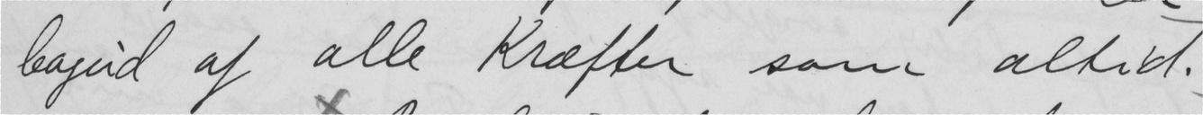bagud af alle Kræfter som altid.
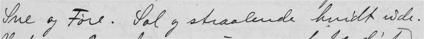Sne og Føre. Sol og straalende hvidt ude.
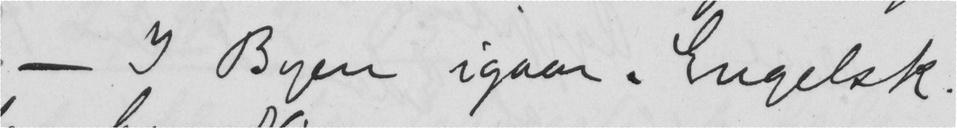- I Byen igaar. Engelsk.
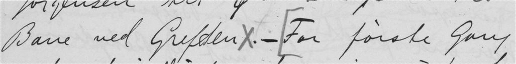Bane ved Greften. For første Gang.
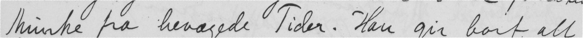Munke fra hevægede Tider. Han gir bort alt
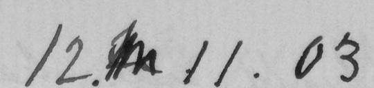12.  11.03
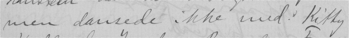men dansede ikke med. Kifty
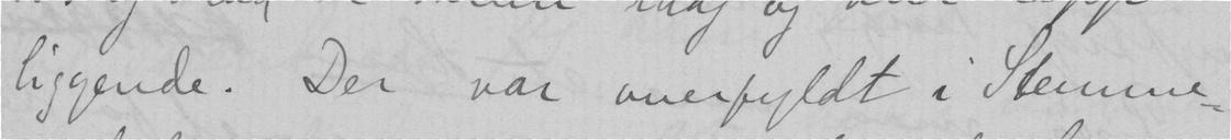liggende. Der var overfyldt i Stemme-
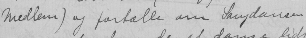medlem) og fortælle om Sangdansen
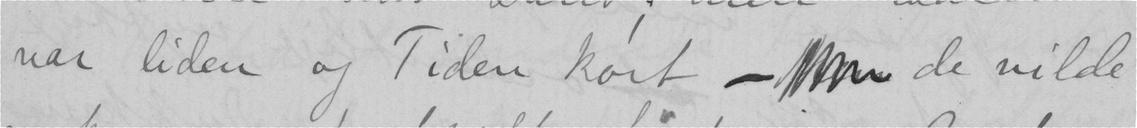var liden og Tiden kort -  de vilde
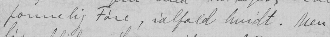formelig Føre, ialfald hvidt. Men
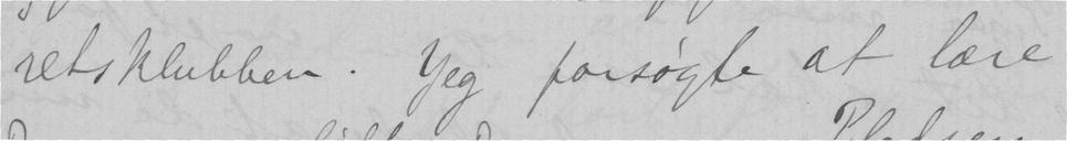retsklubben. Jeg forsøgte at lære
